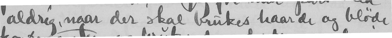aldrig, naar der skal brukes haarde og bløde
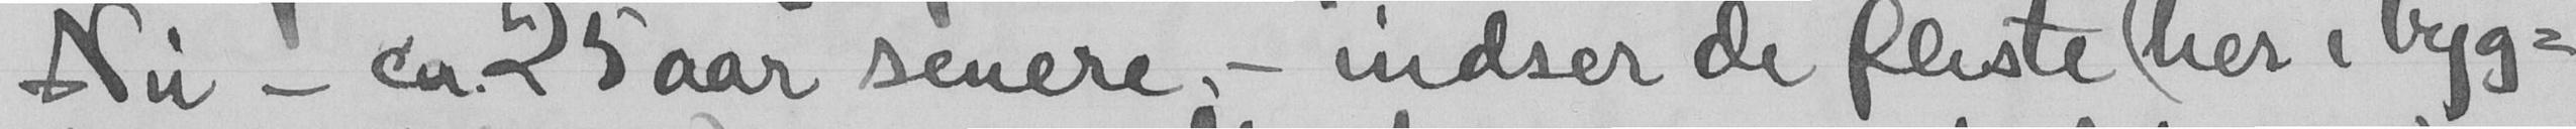Nu - ca 25 aar senere, - indser de fleste (her i byg-
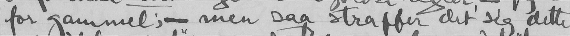for gammel; - men saa straffer det sig dette
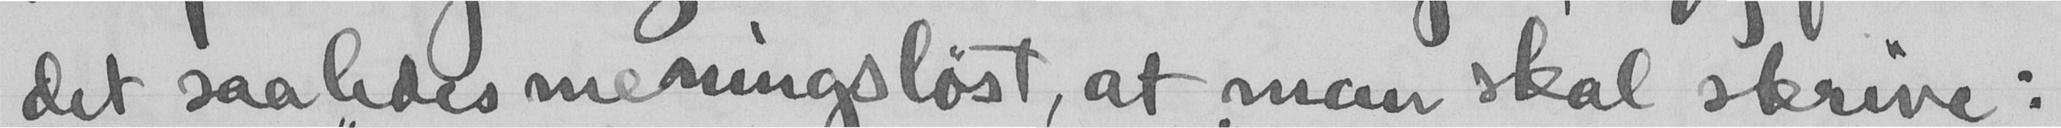det saaledes meningsløst, at maa skal skrive:
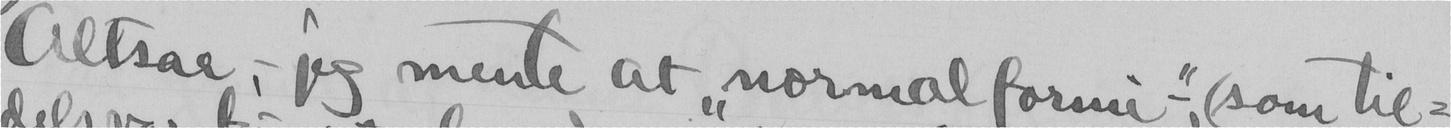Altsaa, - jeg mente at "normalformi", (som til-
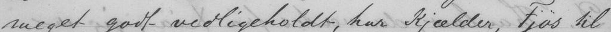meget godt vedligeholdt, har Kjælder, Fjøs til
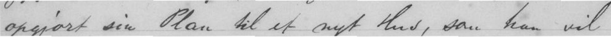opgjort sin Plan til et nyt Hus, som han vil
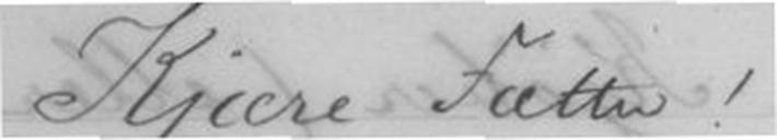Kjære Fætter!
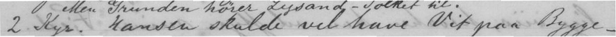2 Kyr. Hansen skulde vel have Vit paa Bygge-
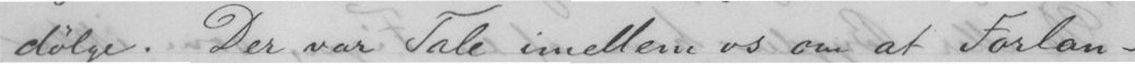dølge. Der var Tale imellem os om at Forlan-
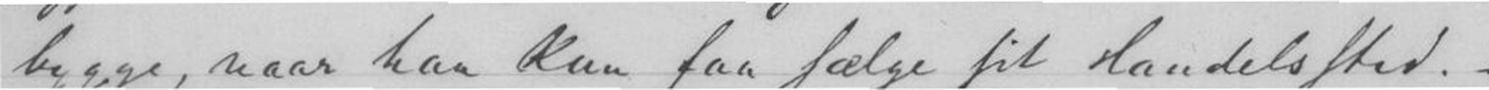bygge, naar han kan faa sælge sit Handelssted. -
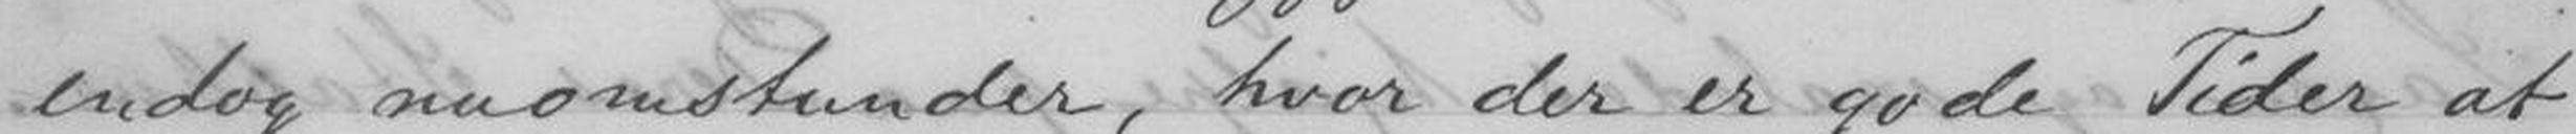endog nu omstunder, hvor der er gode Tider at
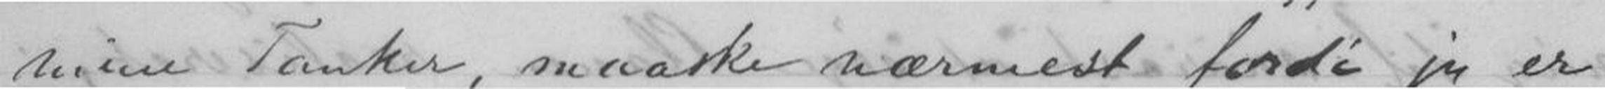mine Tanker, maaske nærmest fordi jeg er
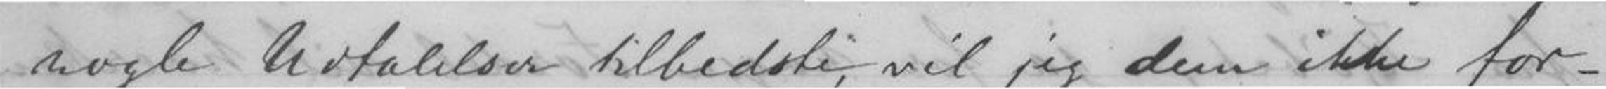nogle Udtalelser tilbedste, vil jeg dem ikke for-
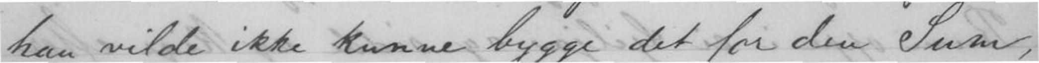han vilde ikke kunne bygge det for den Sum,
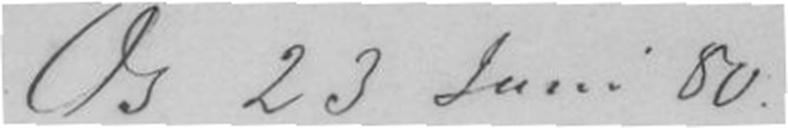Os 23 Juni 80
